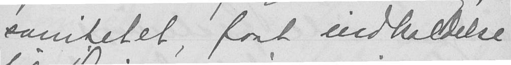sanitetet, faat indkaldelse
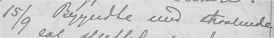15/9 Begyndte med straalende
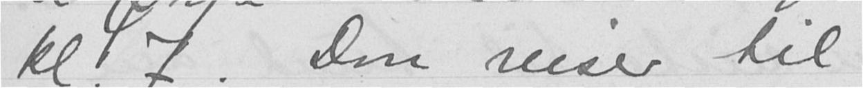kl. 7. Don reiser til
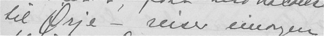til Ørje - reiser imorgen
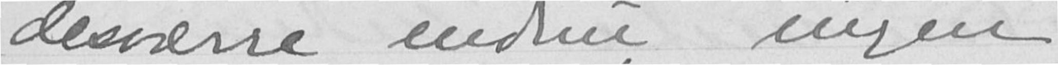desværre endnu ingen
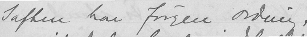Iaften har Jørgen Ording,
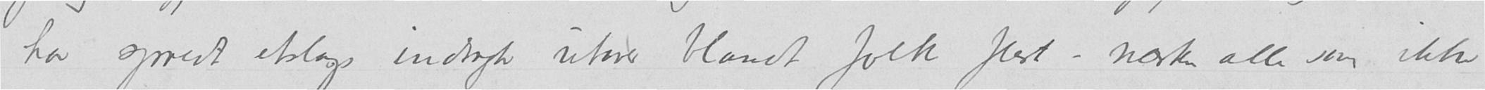har spredt etslags indtryk utover blandt folk flest – næsten alle som ikke
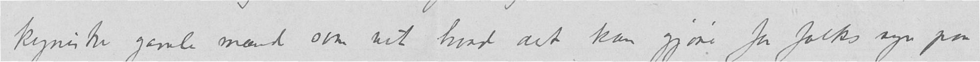kyniske gamle mænd som vet hvad det kan gjøre for folks syn paa
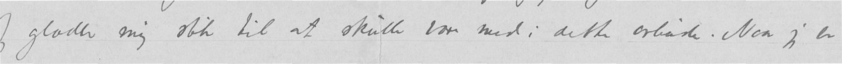Jeg glæder mig slik til at skulle være med i dette arbeide. Naar jeg er
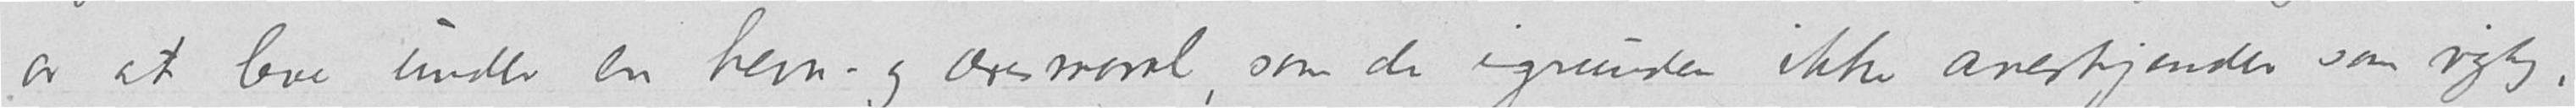av at leve under en hevn- og æresmoral, som de igrunden ikke anerkjender som rigtig,
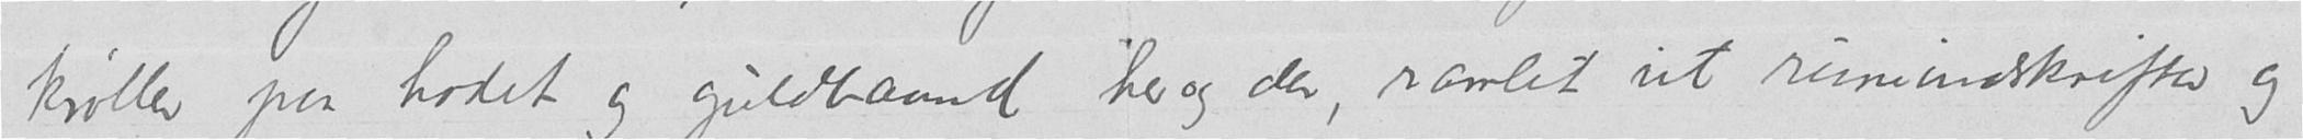krøller paa hodet og guldhaand her og der, ramlet ut rimindskrifter og
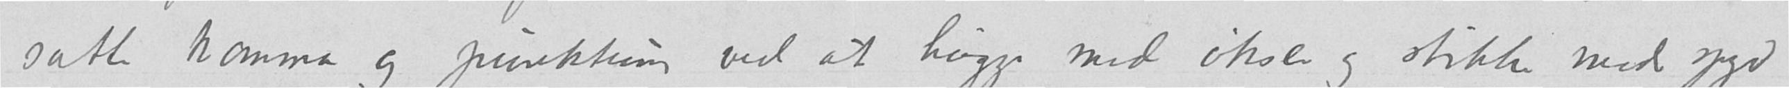satte komma og punktum ved at hugge med økser og stikke med spyd
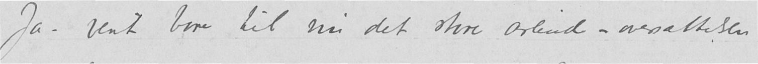Ja – vent bare til nu det store arbeide – oversættelsen
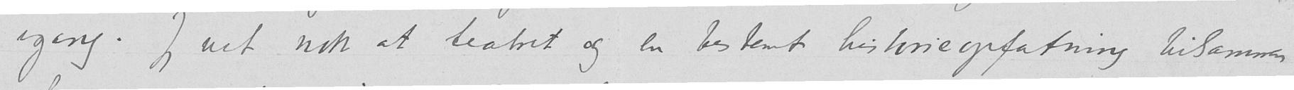igang. Jeg vet nok at teatret og en bestemt historieopfatning tilsammen
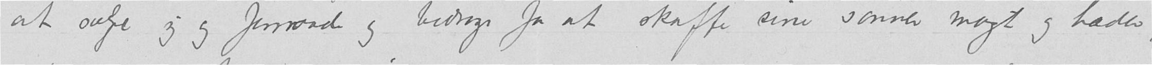at sælge sig og forrraade og bedrage for at skaffe sine sønner magt og hæder,
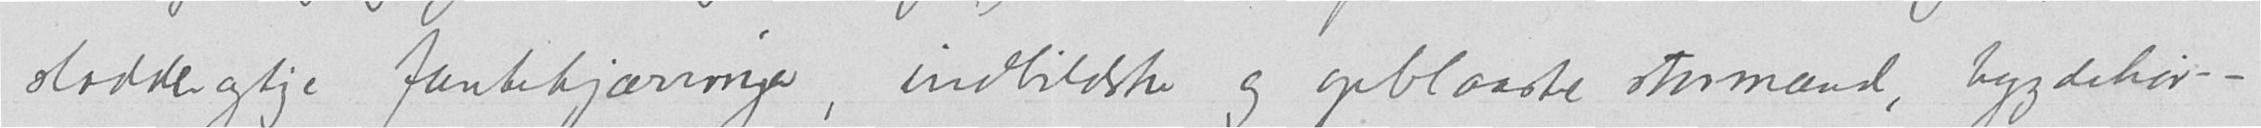sladderagtige fantekjærringer, indbildske og opblaaste stormænd, bygdehøv-
